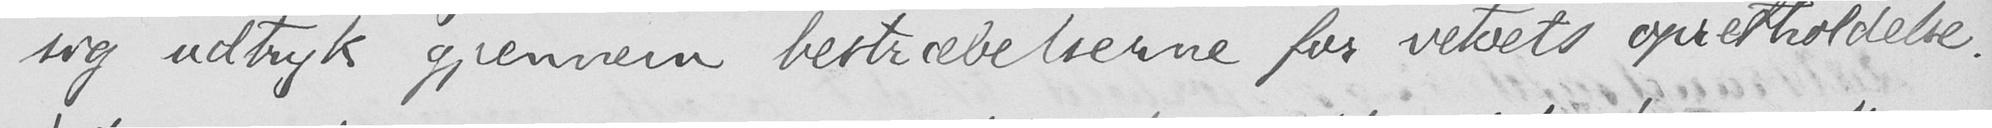sig udtryk gjennem bestræbelserne for vetoets opretholdelse.
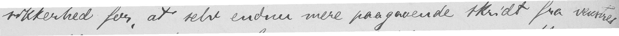sikkerhed for, at selv endnu mere paagaaende skridt fra venstres
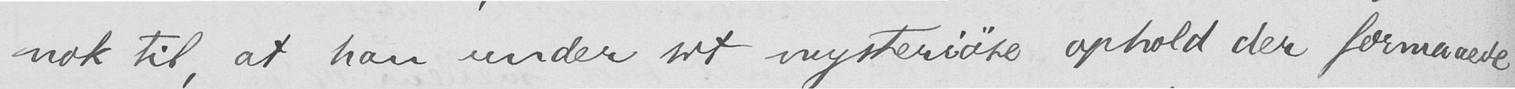nok til, at han under sit mysteriøse ophold der formaaede
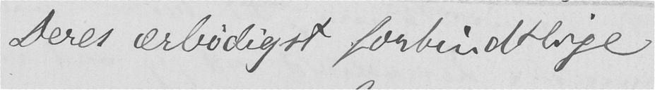Deres ærbødigst forbindtlige
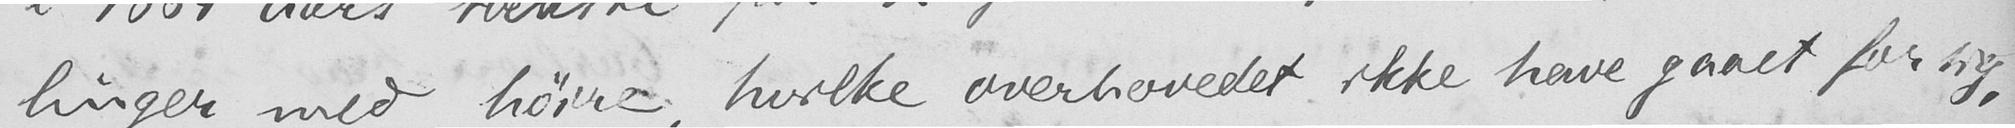linger med høire, hvilke overhovedet ikke have gaaet for sig,
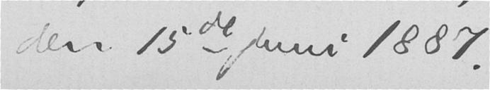den 15de juni 1887.
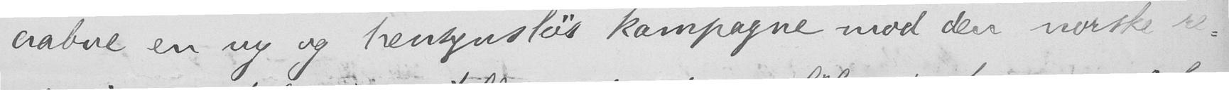aabne en ny og hensynsløs kampagne mod den norske re-
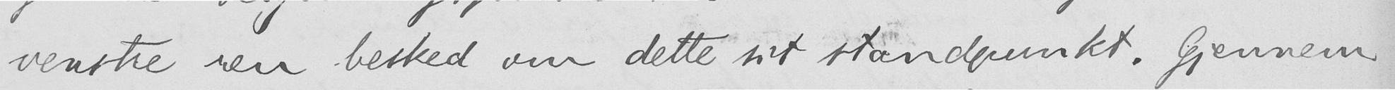venstre ren besked om dette sit standpunkt. Gjennem
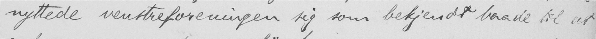nyttede venstreforeningen sig som bekjendt baade til at
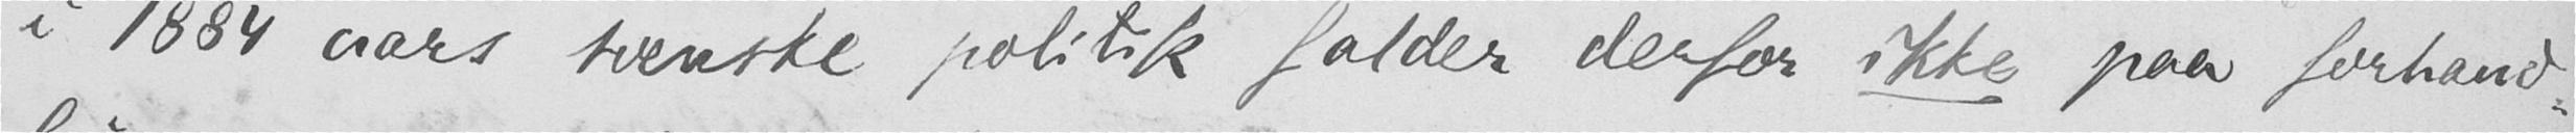i 1884 aars svenske politik falder derfor ikke paa forhand-
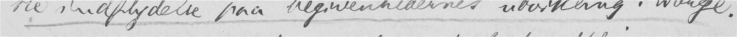ste indflydelse paa begivenhedernes udvikling i Norge.
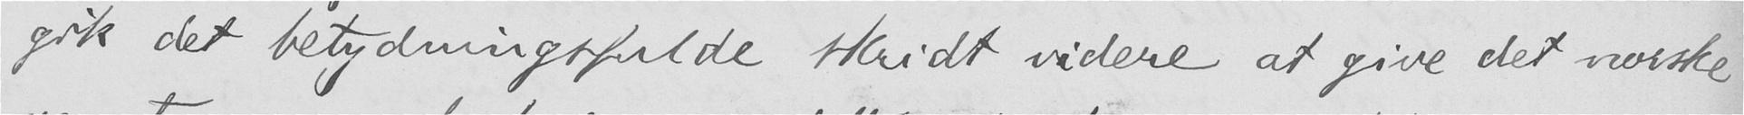gik det betydningsfulde skridt videre at give det norske
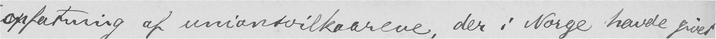opfatning af unionsvilkaarene, der i Norge havde givet
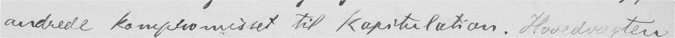andrede kompromisset til kapitulation. Hovedvægten
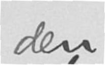den
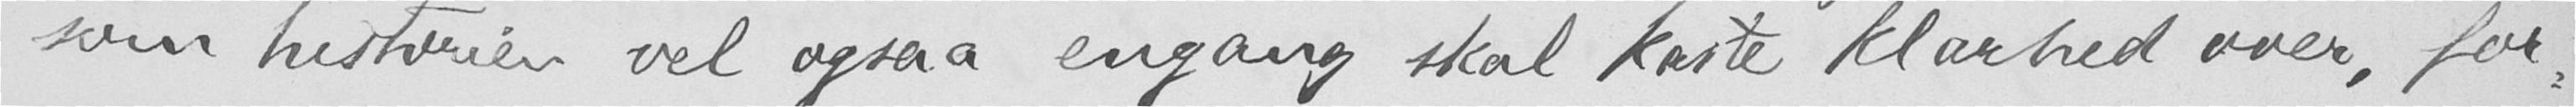som historien vel ogsaa engang skal kaste klarhed over, for-
